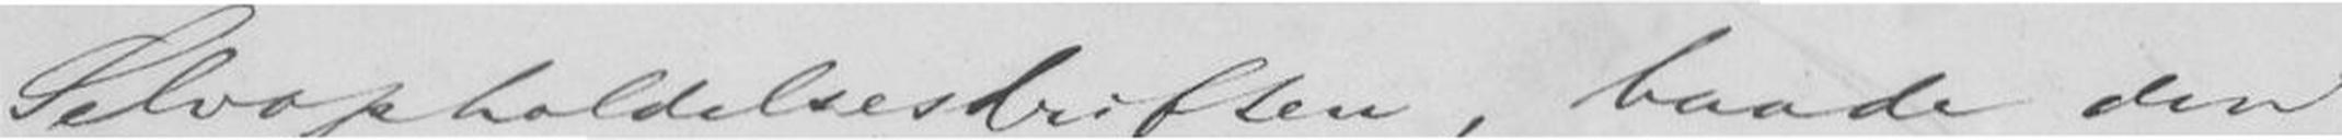Selvopholdelsesdriften, baade den
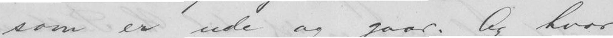som er ude og gaar. Og hvor
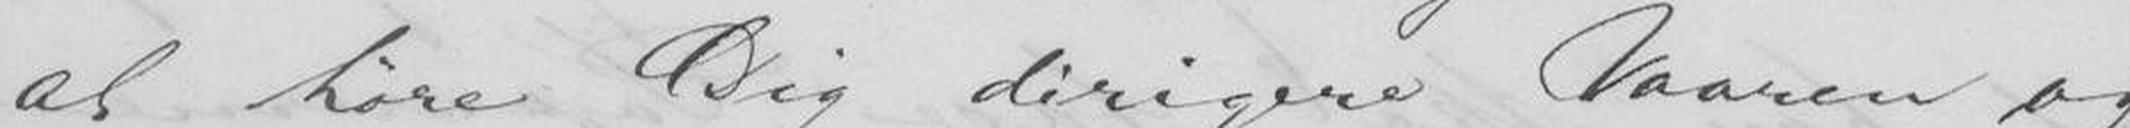at høre Dig dirigere Vaaren og
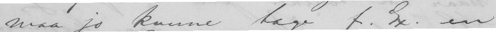maa jo kunne tage f. Ex. en
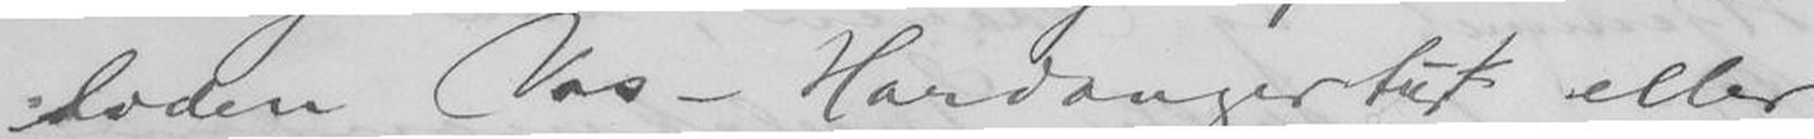liden Vos - Hardangertur eller
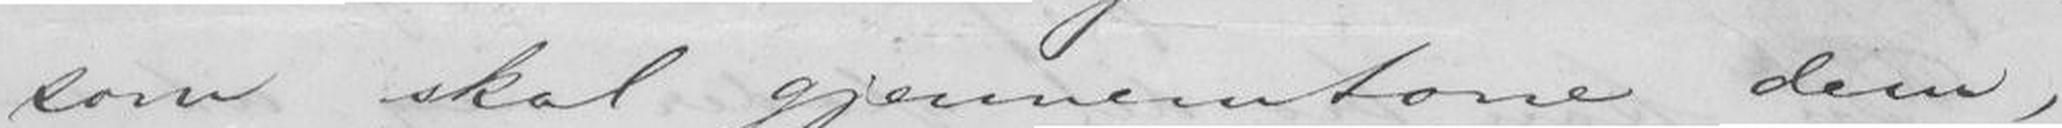som skal gjennemtone dem,
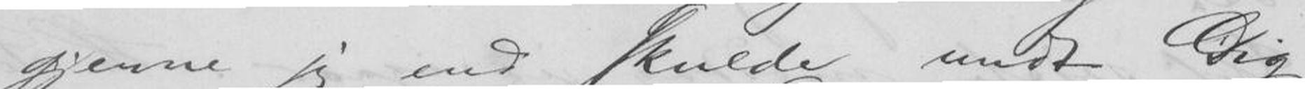gjerne jeg end skulde undt Dig
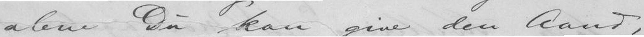alene Du kan give den Aand,
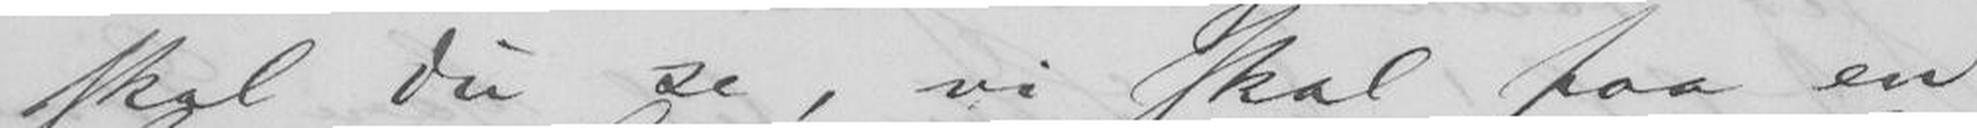skal Du se, vi skal faa en
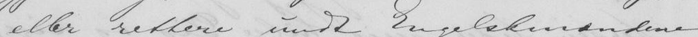eller rettere undt Engelskmændene
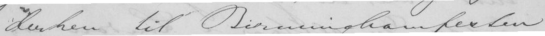til Birminghamfesten
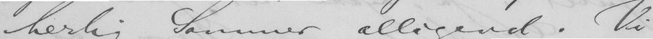herlig Sommer alligevel. Vi
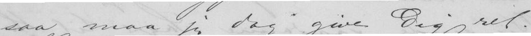saa maa jeg dog give Dig ret.
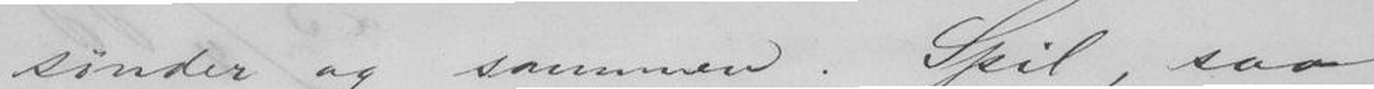sønder og sammen. Spil, saa
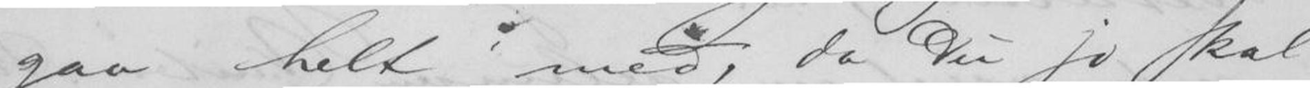gaa helt med, da Du jo skal
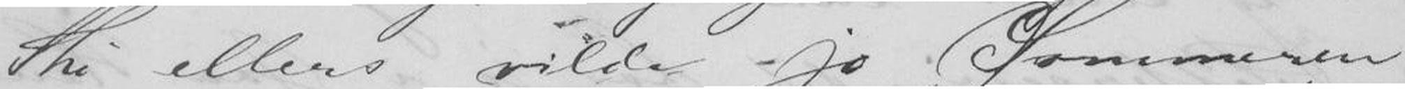Thi ellers vilde jo Sommeren
